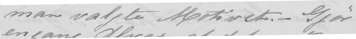man valgte Motivet. - Gjør
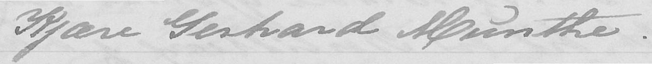Kjære Gerhard Munthe.
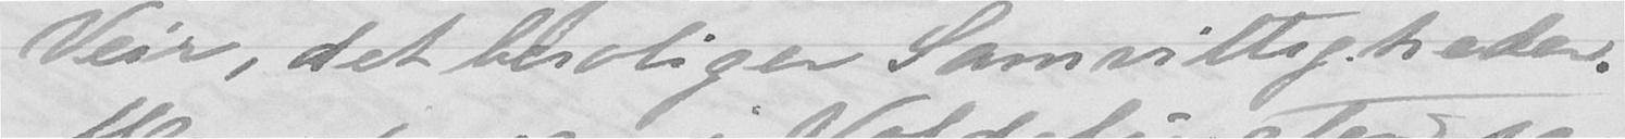Veir, det beroliger Samvittigheden.
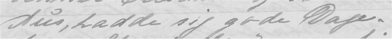Aus, hadde sig gode Dage.
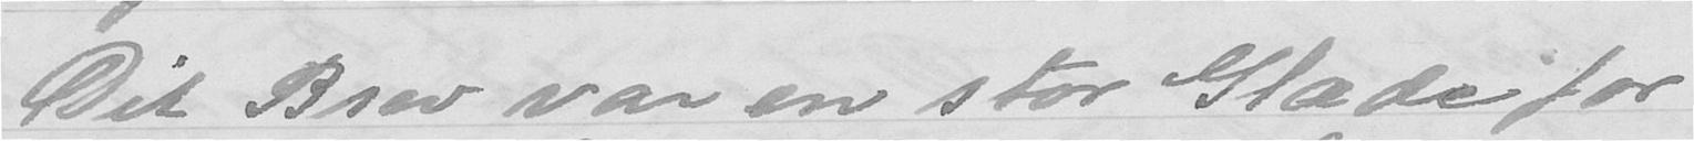Dit Brev var en stor Glæde for
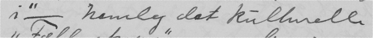i" – nemleg det kulturelle
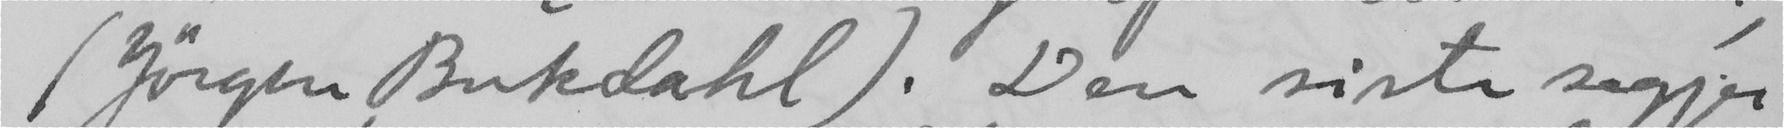(Jörgen Bukdahl). Den siste segjer
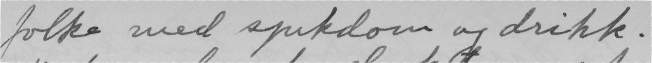folke med sjukdom og drikk.
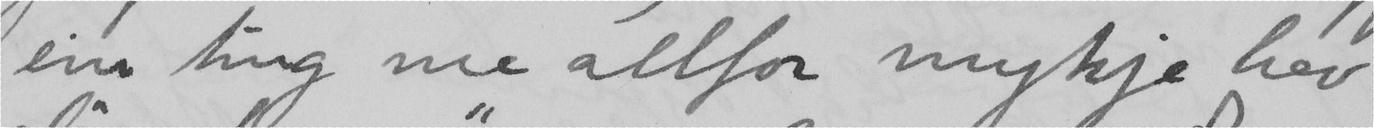ein ting me altfor mykje hev
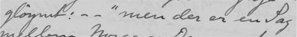glöymt; – "men der er en Sag
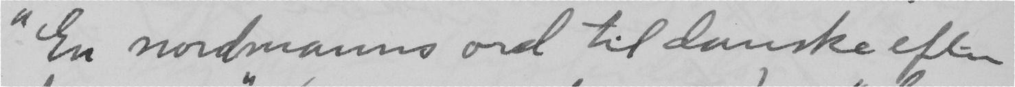"En nordmanns ord til danske efter
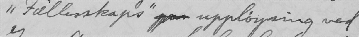"Fællesskaps"  upplöysing ved
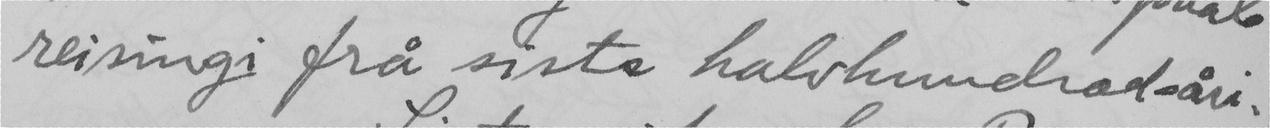reisingi frå siste halvhundrad-åri.
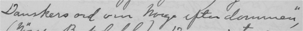Danskers ord om Norge efter dommen",
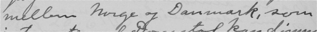mellem Norge og Danmark, som
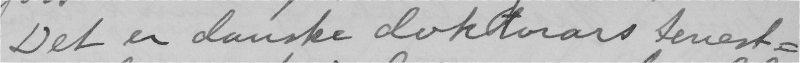Det er danske doktorars tenest-
